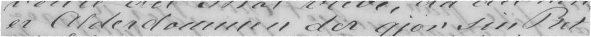er Alderdommen der gjør sin Ret
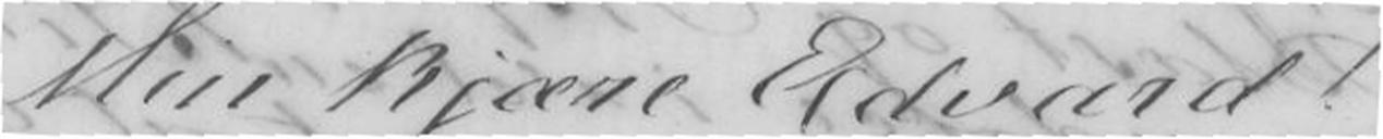Min kjære Edvard!
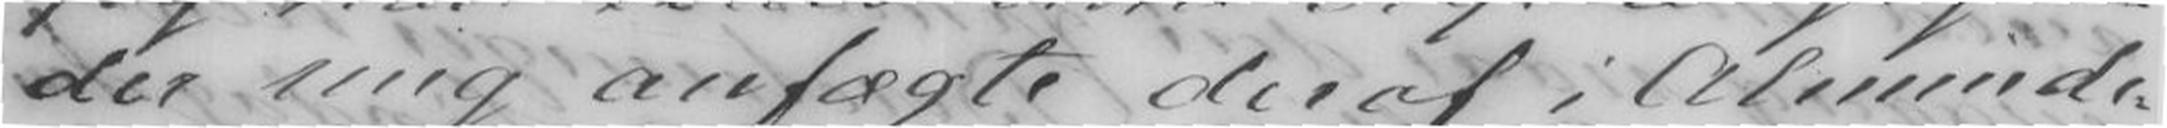der mig anfægte deraf i Alminde-
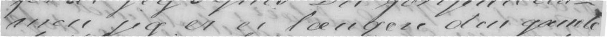men jeg er ei længere den gamle
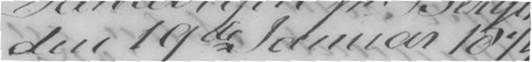den 19de Januar 1874
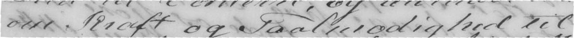om Kraft og Taalmodighed til
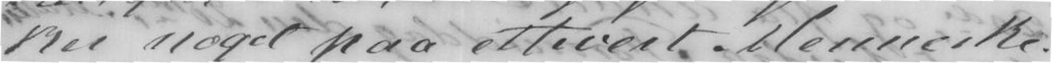ker noget paa ethvert Menneske.
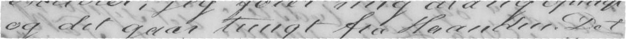og det gaar tungt fra Haanden. Det
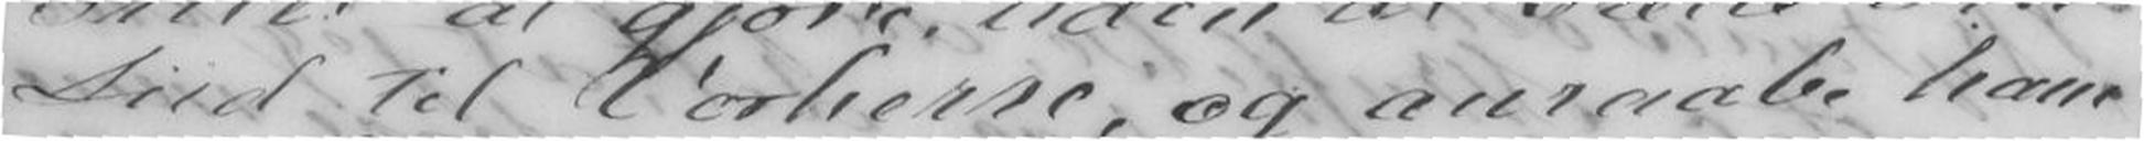Liid til Vorherre, og anraabe ham
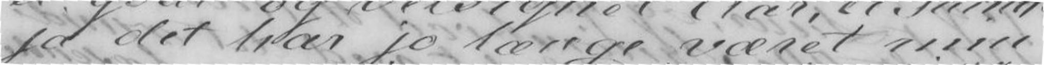ja det har jo længe været min
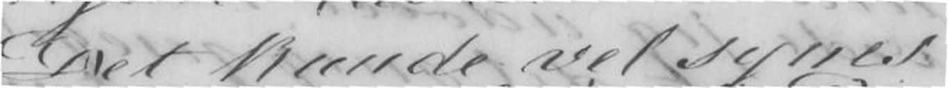Det kunde vel synes
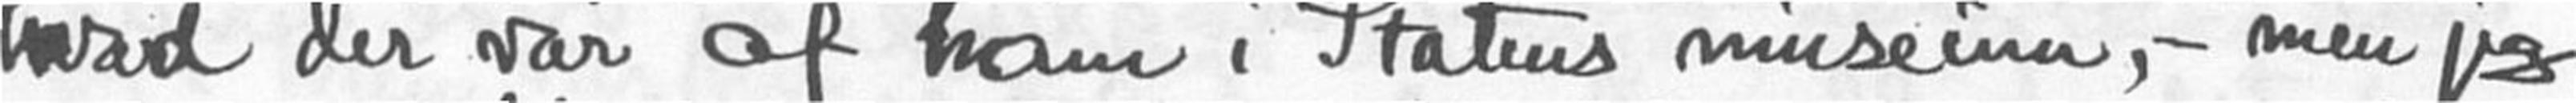hvad der var af ham i Statens museum, - men jeg
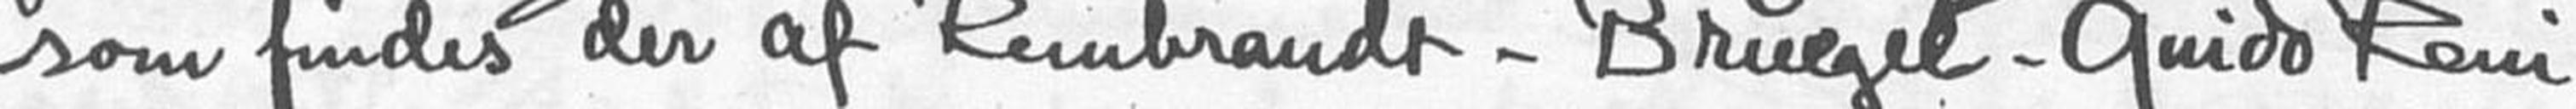som findes der af Rembrandt - Bruegel - Guido Reni -
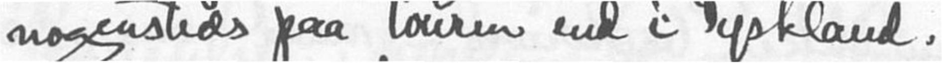nogensteds paa touren end i Tyskland.
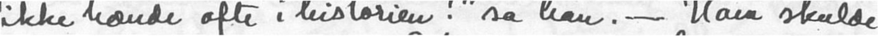ikke hænde ofte i historien !" sa han. - Han skulde
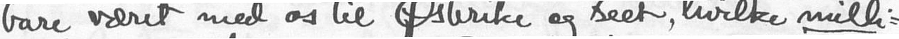bare været med os til Østerike og seet, hvilke milli-
